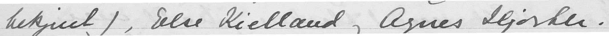bekjent), Else Kielland og Agnes Hjort.
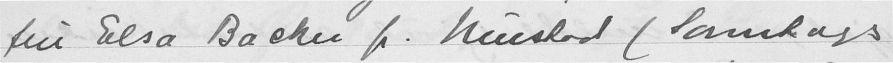fru Elsa Backer fr. Mustad (Sorentags
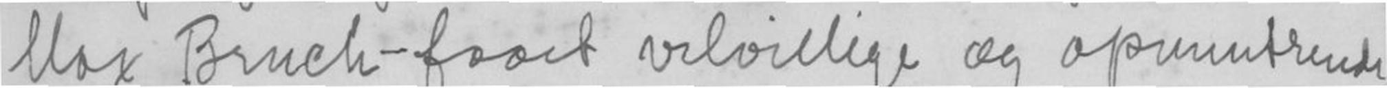Max Bruch - faaet velvillige og opmuntrende
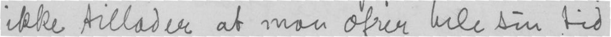ikke tillader at man ofrer hele sin tid
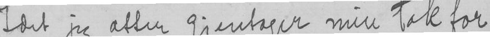Idet jeg atter gjentager min Tak for
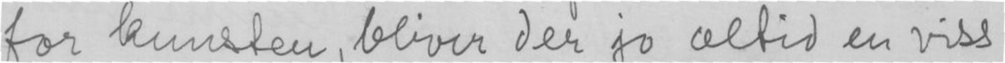for kunsten, bliver der jo altid en viss
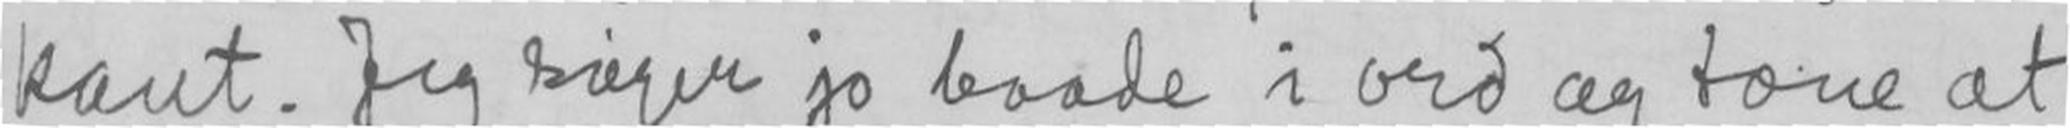kant. Jeg søger jo baade i ord og tone at
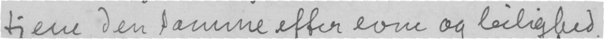tjene den samme efter evne og leilighed.
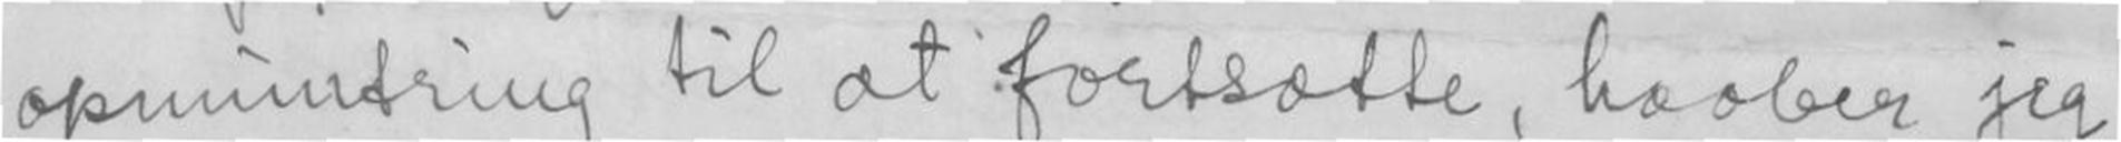opmuntring til at fortsætte, haaber jeg
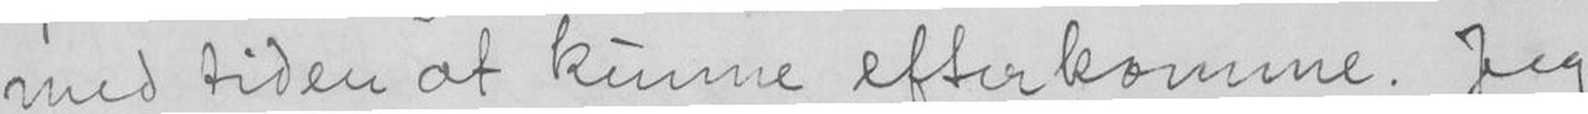med tiden at kunne efterkomme. Jeg
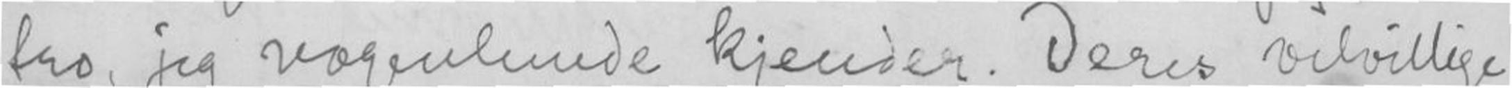tro jeg nogenlunde kjender. Deres velvillige
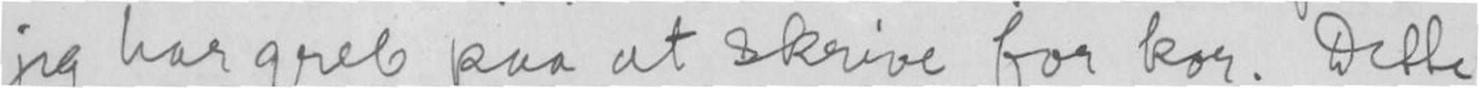jeg har greb paa at skrive for kor. Dette
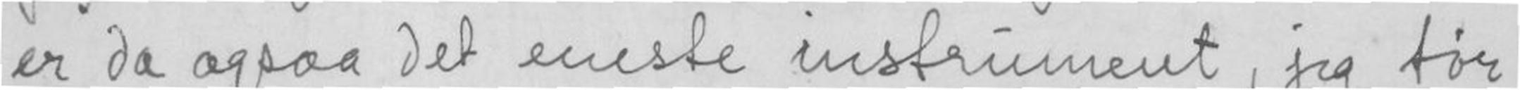er da ogsaa det eneste instrument, jeg tør
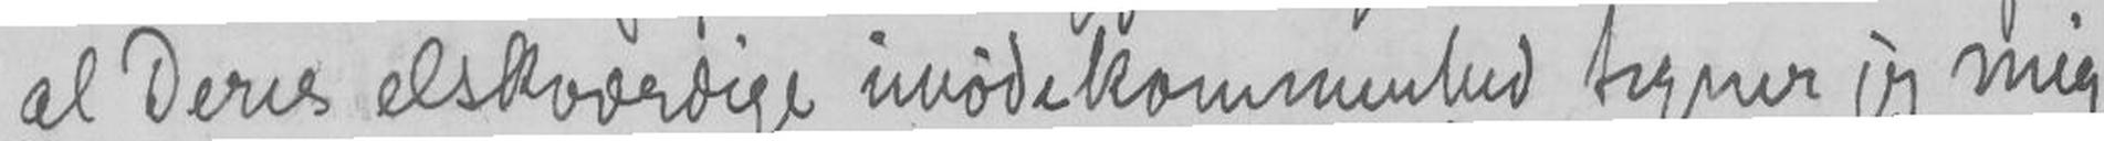al Deres elskværdige imødekommenhed tegner jeg mig
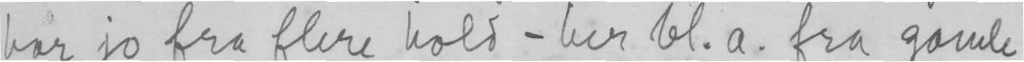har jo fra flere hold - her bl.a. fra gamle
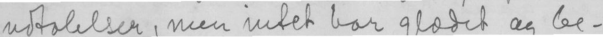udtalelser, men intet har glædet og be-
